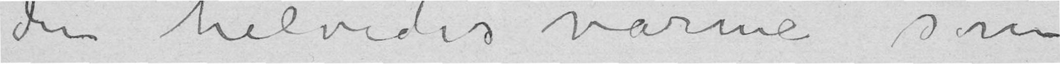den helvedes varme som
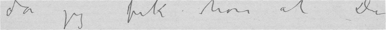da jeg fik høre at De
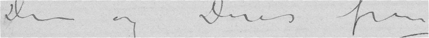dem og Deres frue –
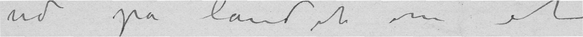ud på landet om et
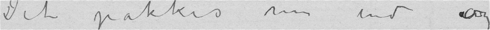Det pakkes nu ind og
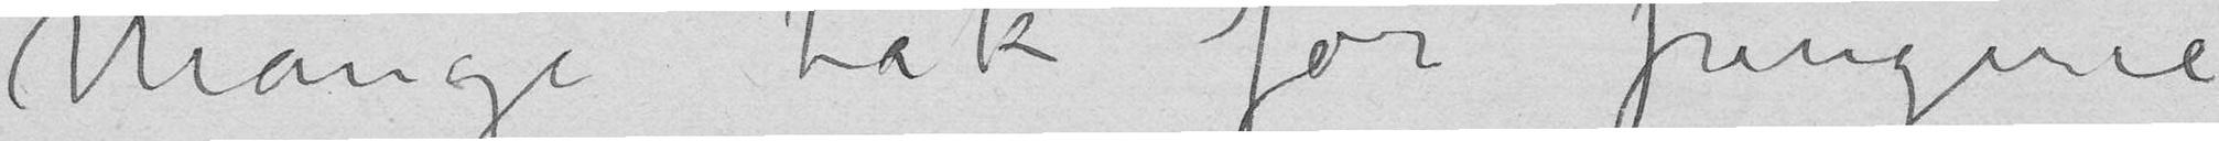Mange tak for pengene
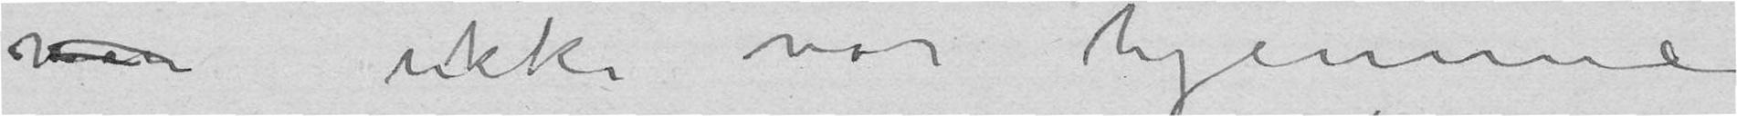ikke var hjemme –
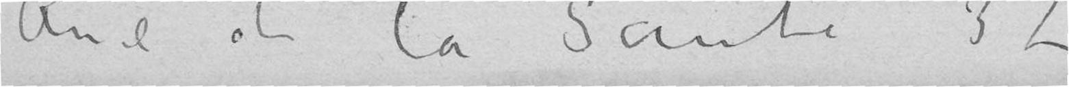Rue de la Sante 32
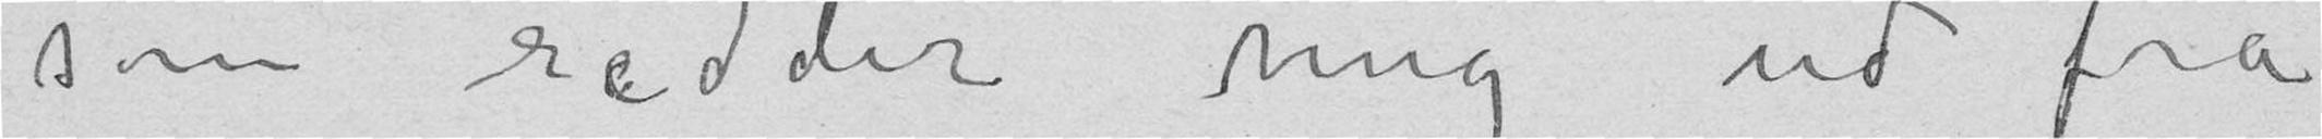som redder mig ud fra
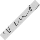Vend
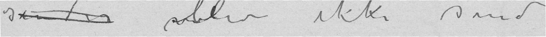sendes ble ikke sendt
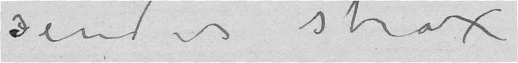sendes strax –
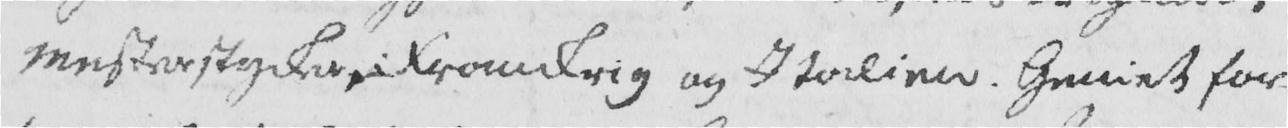Mesterstycker, i Frankrig og Italien. Geniet for-
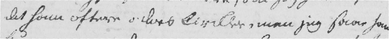det ham oftere i deres Cirkler, men jeg saae ham
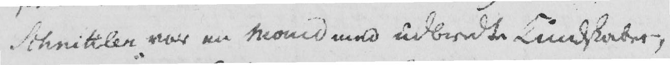Schnittler var en Mand med udbredte Kundskaber,
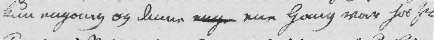kun engang og denne  ene Gang var hos Hr
